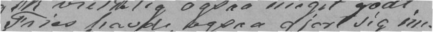Fries havde ogsaa meget gjort sig im-
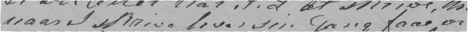naar I dkrive hver sin Gang faae vi
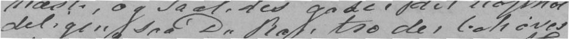deligen saa Du kan tro der behøves
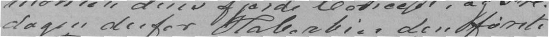dagen derfor Haberbier den første
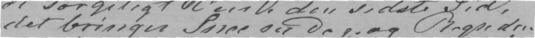det bringer Snee en Dag, og Regn den
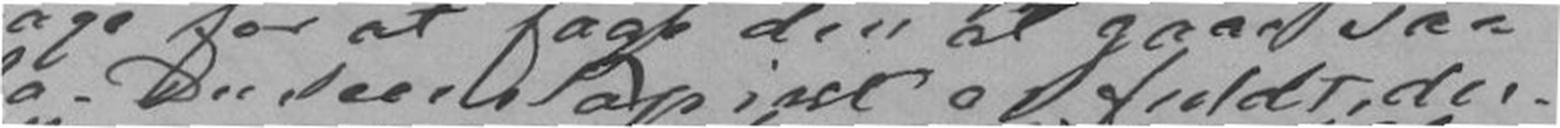Du seer Papiret er fuldt, der
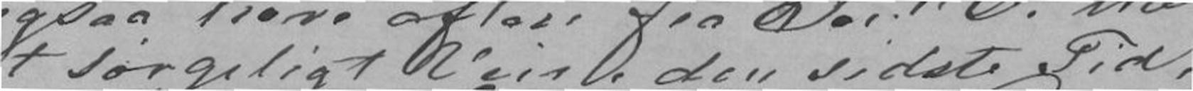et sørgeligt Veir den sidste Tid,
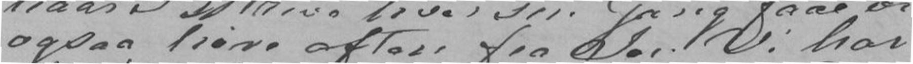ogsaa høre oftere fra Jer. Vi har
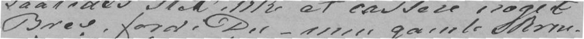Brev, fordi Du - min gamle skrue,
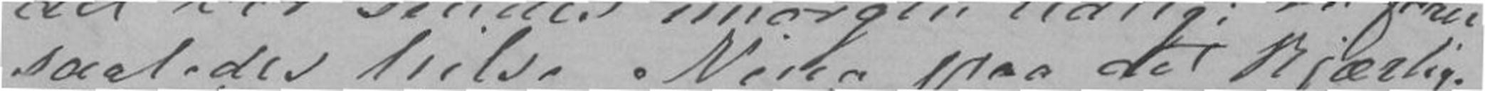saaledes hilse Nina paa det kjærlig-
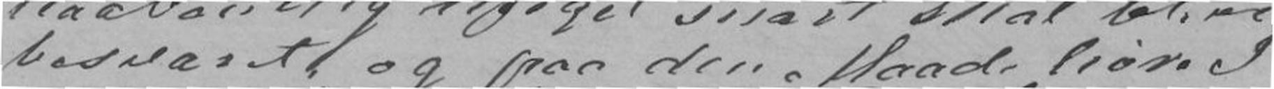besvaret, og paa den Maade høre I
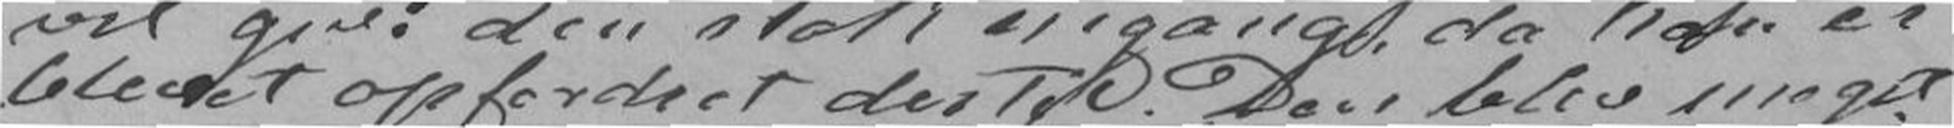blevet opfordret dertil. Den blev meget
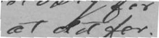at det for-
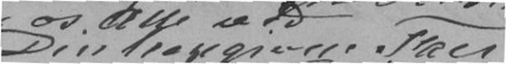Din hengivne Faer
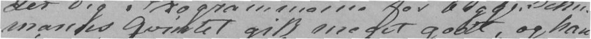manns Qvintet gik meget godt, og han
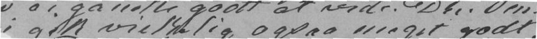gik virkelig ogsaa megget godt,
